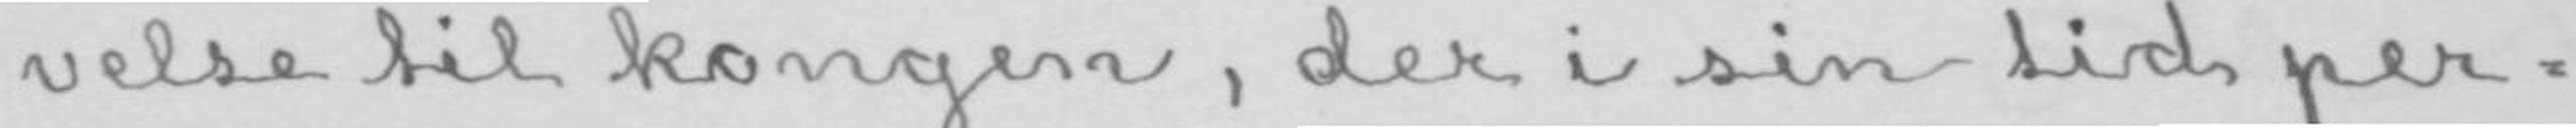velse til kongen, der i sin tid per-
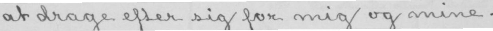at drage efter sig for mig og mine.
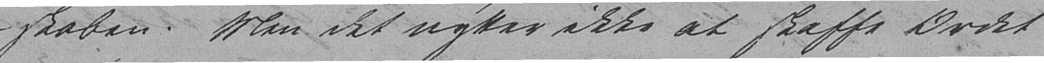skaben. Men det nytter ikke at skaffe Ordet
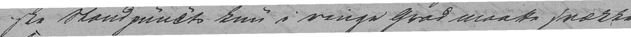ske Standpunkt kun i ringe Grad maatte svække
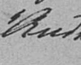And-
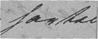har til
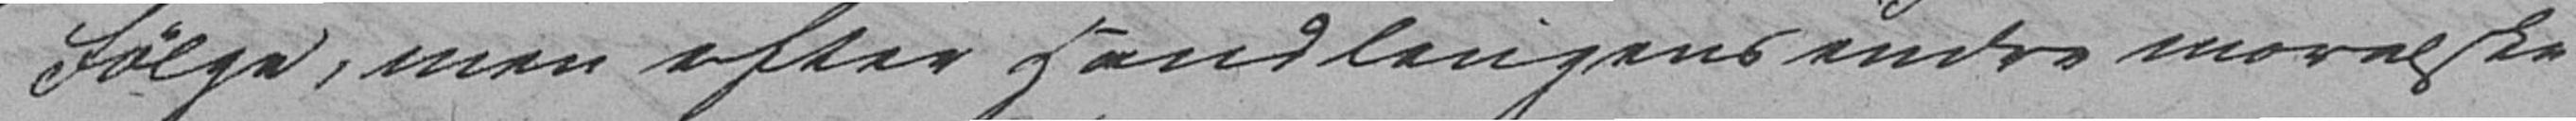Følge, men efter Handlingens indre moralske
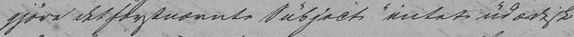gjøre det førstnævnte Subject "intet udædisk
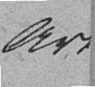An-
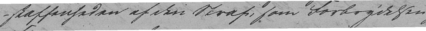skaffenheden af den Straf, som Forbrydelsen
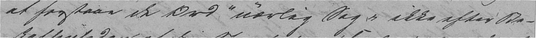at forstaae de Ord "uærlig Sag," ikke efter Be-
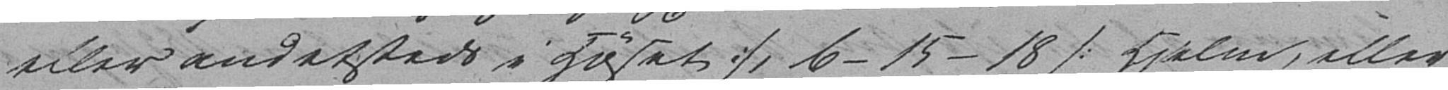eller andetsteds i Huset :/, 6-15-18 /: Hjelm, eller
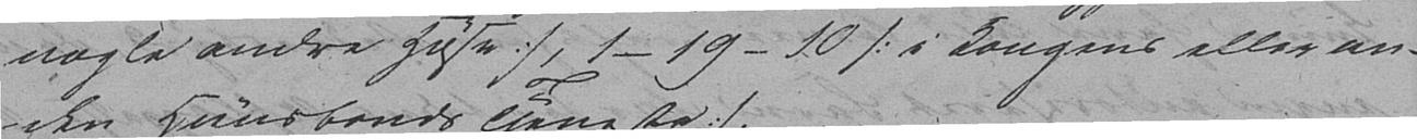nogle andre Huse :/, 1-19-10 /: i Kongens eller an-
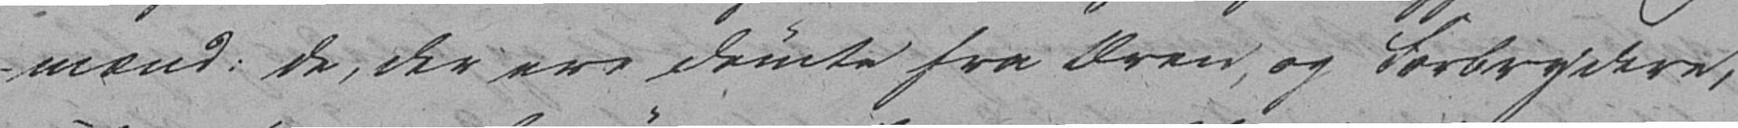mænd: de, der var dømte fra Æren, og forbrydere,
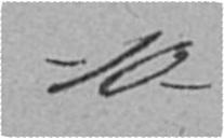- 10 -
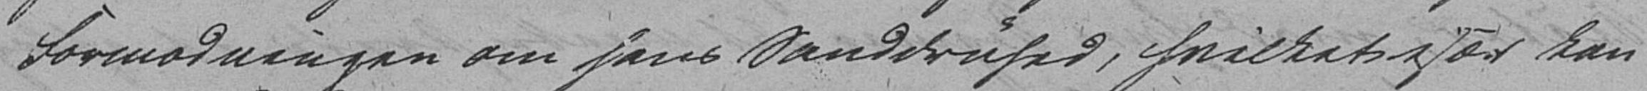Formodningen om hans Sanddruhed, hvilket især kan
